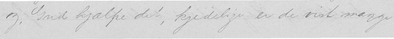og, Gud hjælpe det, kjedelige er de vist mange
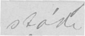støde
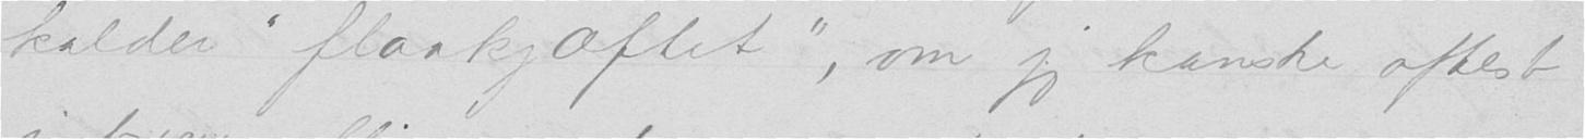kalder "flaakjæftet", om jeg kanske oftest
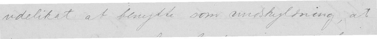udelikat at benytte som undskyldning, at
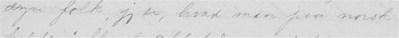ærgre folk, jeg er, hvad man paa norsk
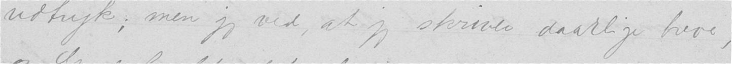udtryk; men jeg ved, at jeg skriver daarlige breve,
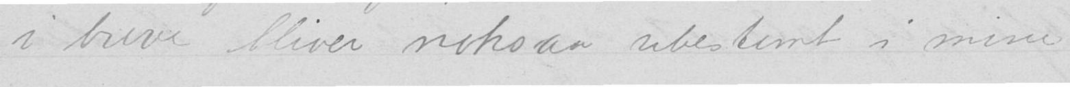i breve bliver noksaa ubestemt i mine
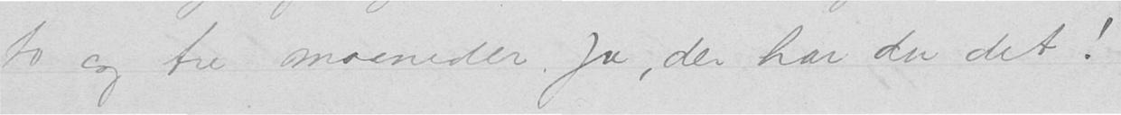to og tre maaneder. Ja, der har du det!
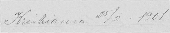Kristiania 25/2-1901
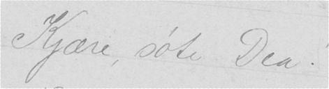Kjære, søte Dea!
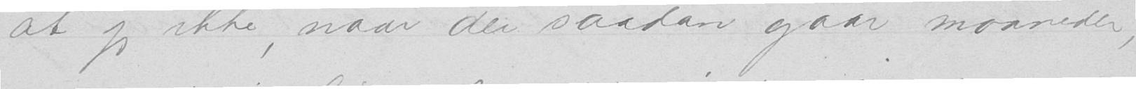at jeg ikke, naar der saadan gaar maaneder,
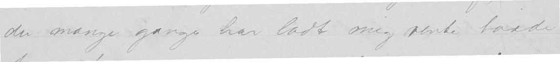du mange gange har ladt mig vente baade
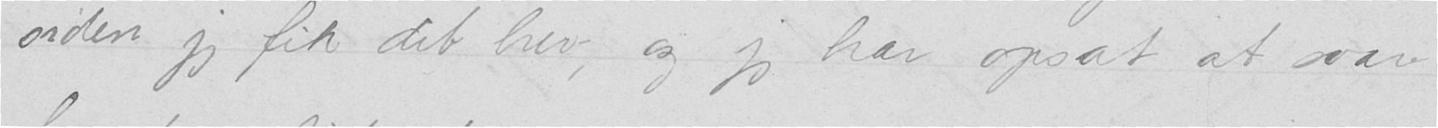siden jeg fik dit brev, og jeg har opsat at svare
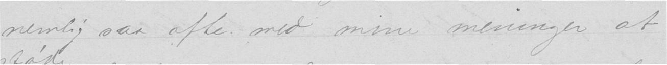nemlig saa ofte med mine meninger at
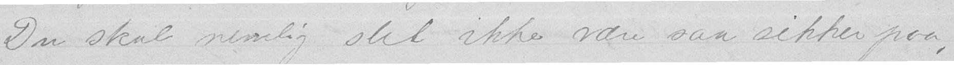Du skal nemlig slet ikke være saa sikker paa,
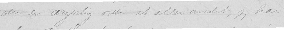du er ærgerlig over et eller andet, jeg har
